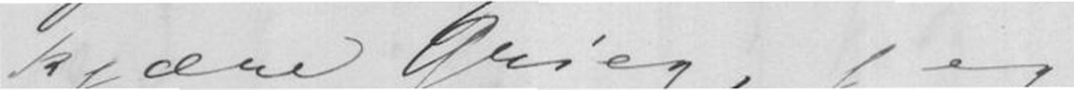kjære Grieg, jeg
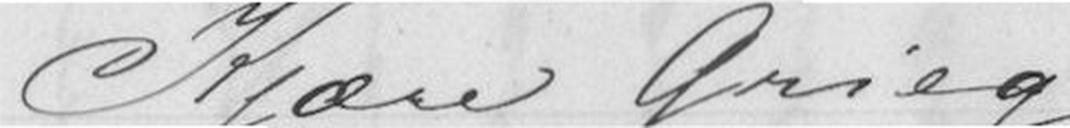Kjære Grieg,
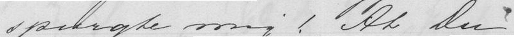spurgte mig! At Du
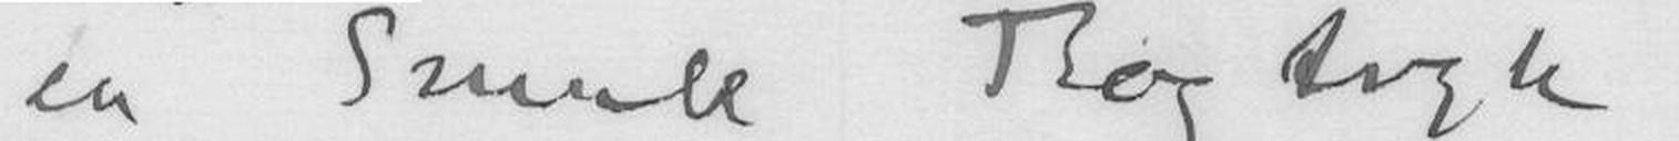en Smuk Bogtryk.
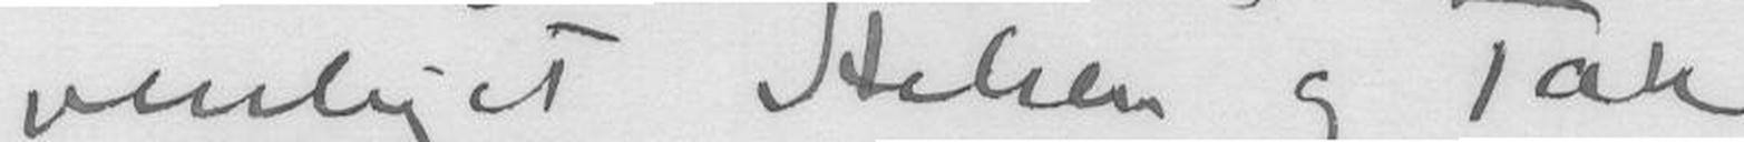venligst Hilsen og Tak
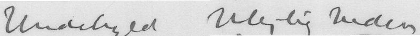Undskyld Ulejligheden
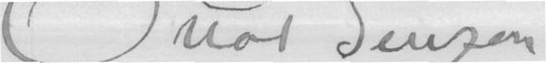Otto Benzon
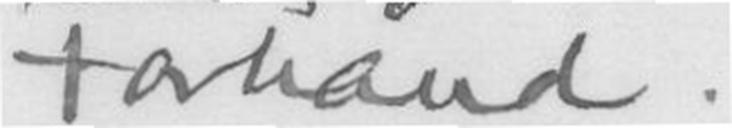Forhånd
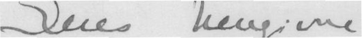Deres hengivne
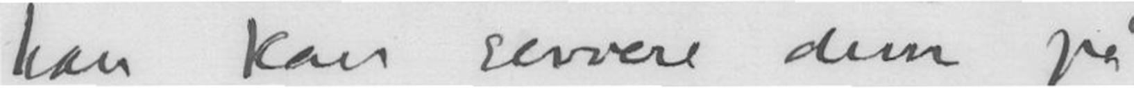han kan servere dem på
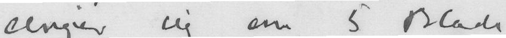drejer sig om 5 Blade
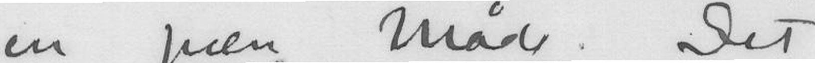en pæn Måde. Det
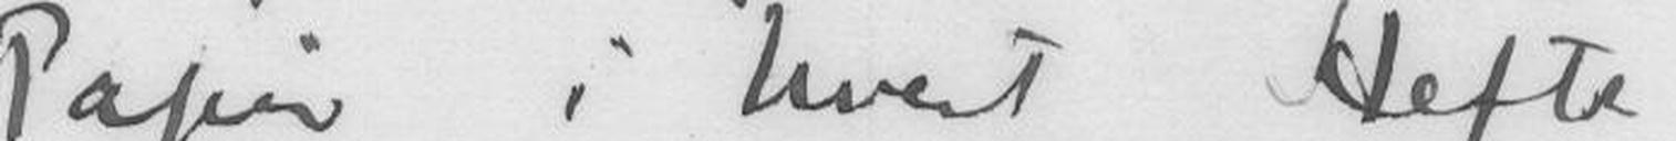Papir i hvert Hefte
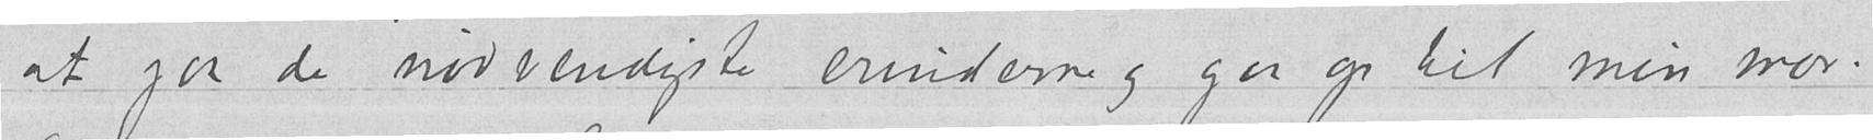at gaa de nødvendigste erinderne og gaa op til min mor.
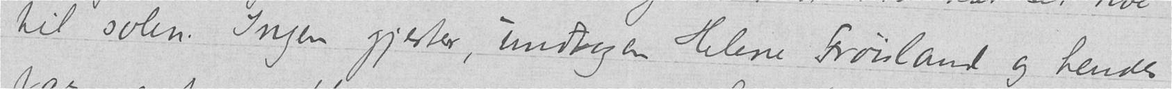til solen. Ingen gjester, undtagen Helene Frøisland og hendes
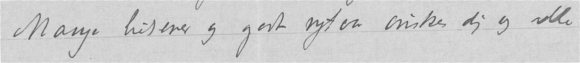Mange hilsener og godt nytaar ønskes dig og alle
